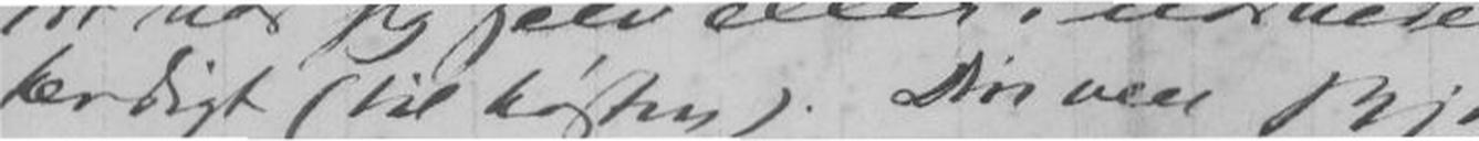færdigt (til høsten).Din ven Bjørn.
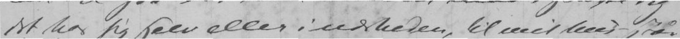det hos sig selv eller i nærheden, til mit bud står
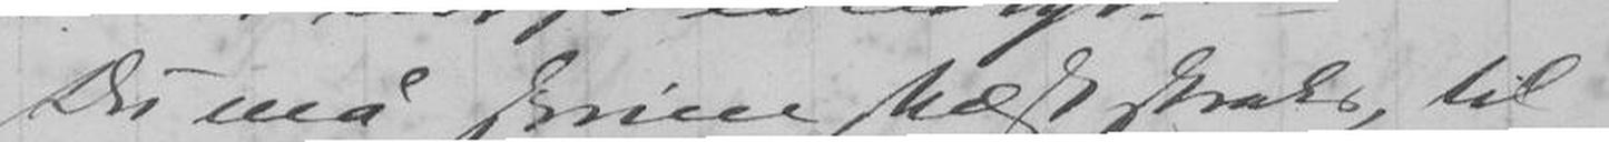Du må skrive mæget straks, til
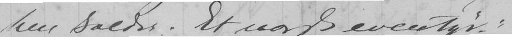hen kalder Et norsk eventyr.
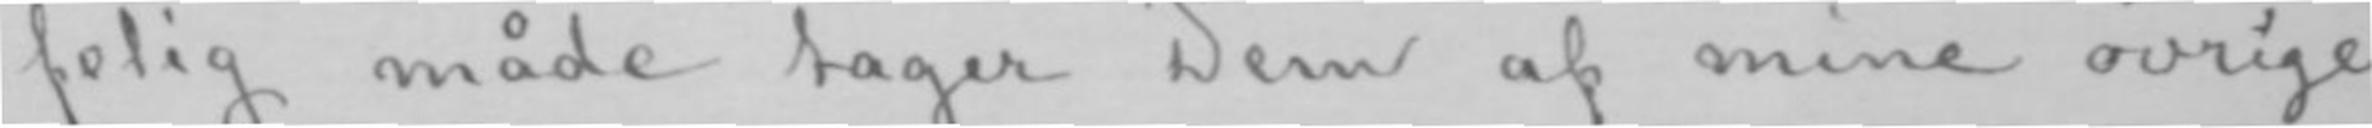felig måde tager Dem af mine øvrige
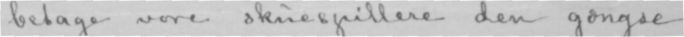betage vore skuespillere den gængse
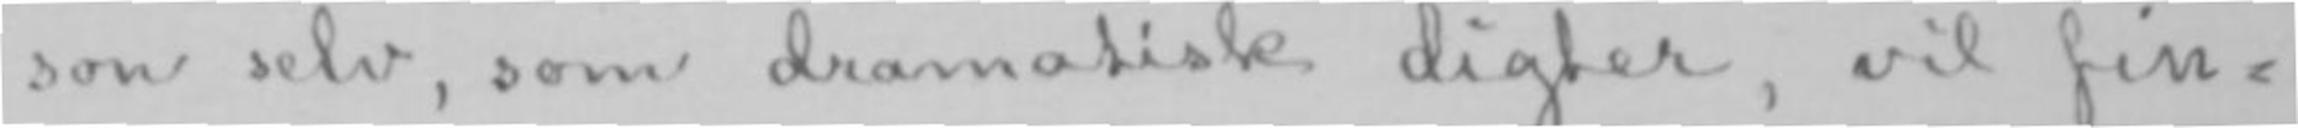son selv, som dramatisk digter, vil fin-
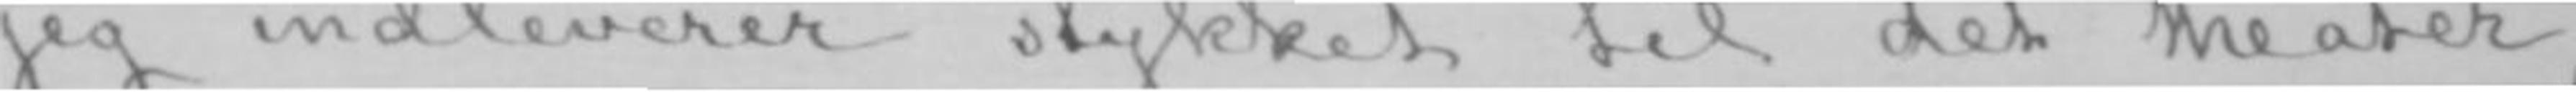jeg indleverer stykket til det theater,
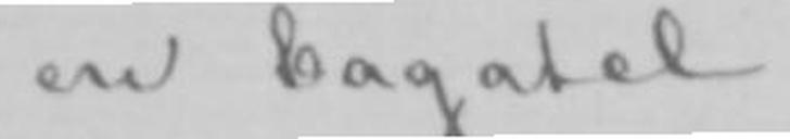en bagatel.-
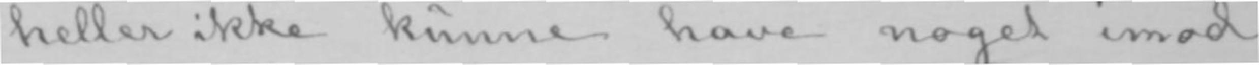heller ikke kunne have noget imod
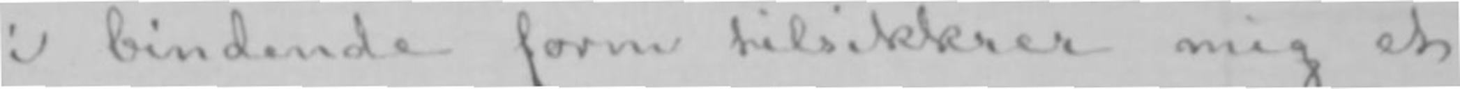i bindende form tilsikkrer mig et
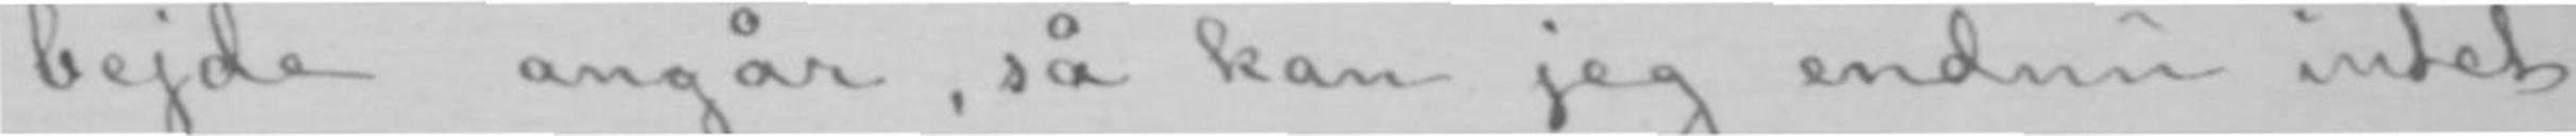bejde angår, så kan jeg endnu intet
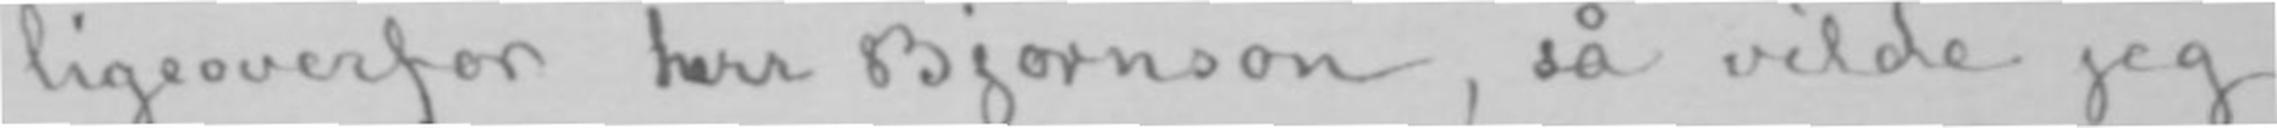ligeoverfor herr Bjørnson, så vilde jeg
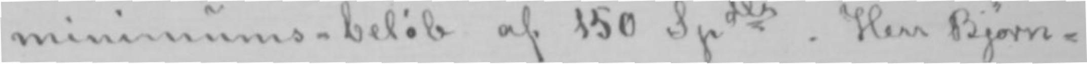minimums-beløb af 150 Spdlr. Herr Bjørn-
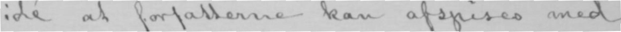idé at forfatterne kan afspises med
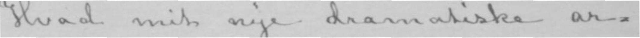Hvad mit nye dramatiske ar-
